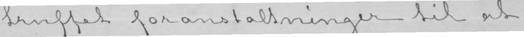truffet foranstaltninger til at
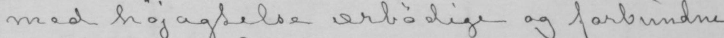med højagtelse ærbødige og forbundne
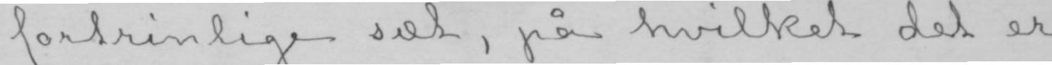fortrinlige sæt, på hvilket det er
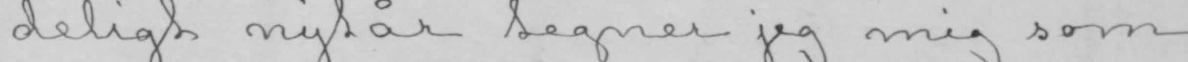deligt nytår tegner jeg mig som
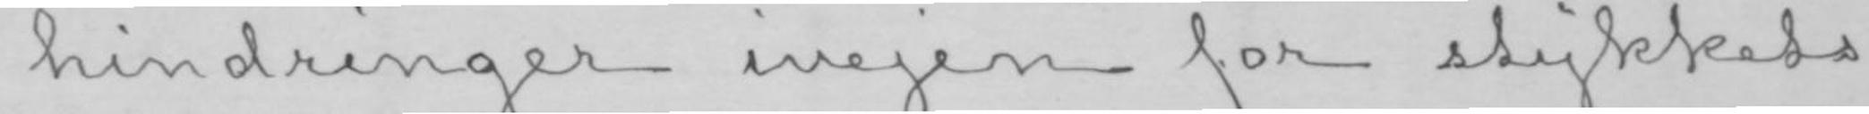hindringer ivejen for stykkets
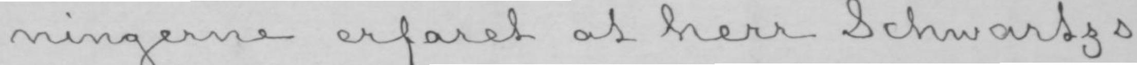ningerne erfaret at herr Schwartzs
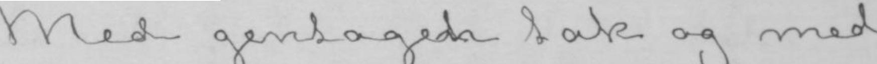Med gentagetn tak og med
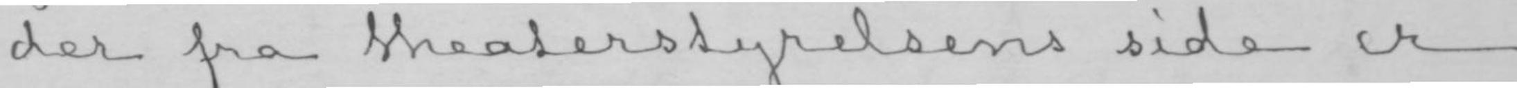der fra theaterstyrelsens side er
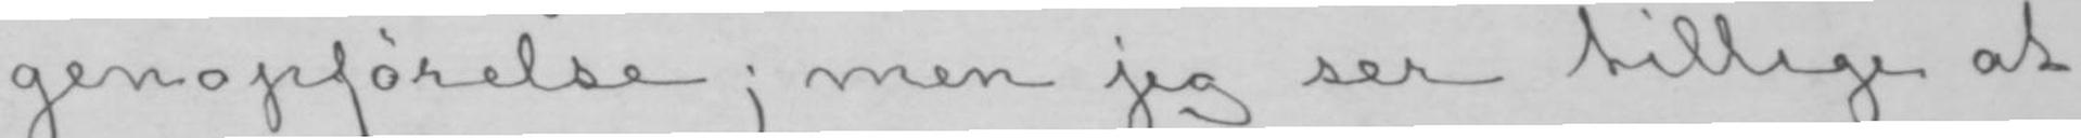genopførelse; men jeg ser tillige at
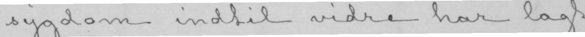sygdom indtil vidre har lagt
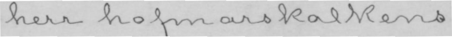herr hofmarskalkens
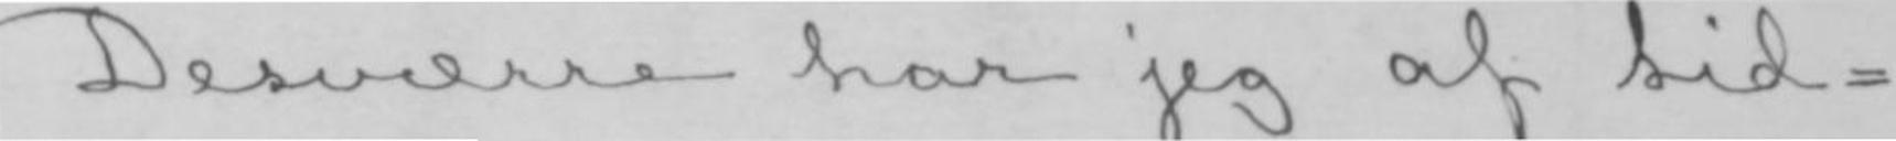Desværre har jeg af tid-
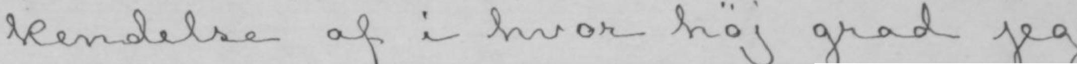kendelse af i hvor høj grad jeg
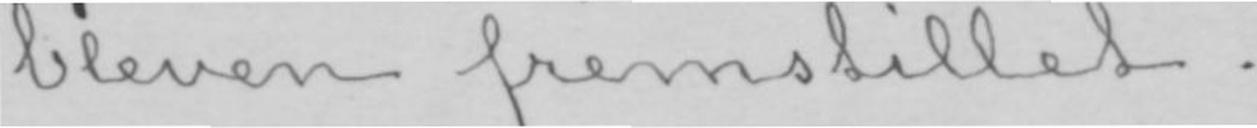bleven fremstillet.
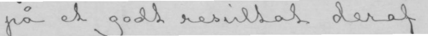på et godt resultat deraf.
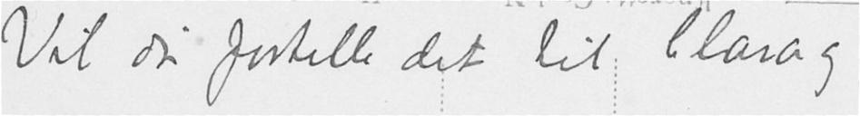Vil du fortelle det til Clara og
